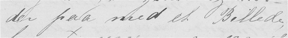der paa med et Billede,
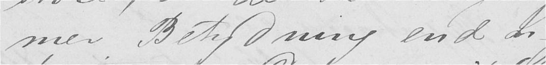mer Betydning end In-
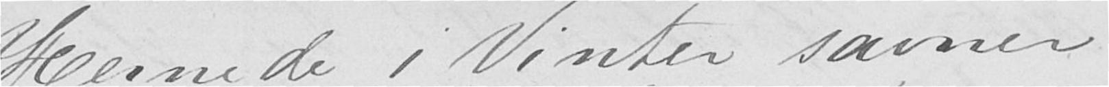Hernede i Vinter savner
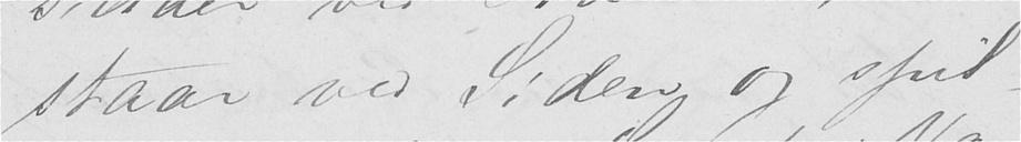staar ved Siden og spil-
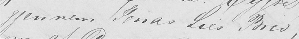gjennem Jonas Lies Brev,
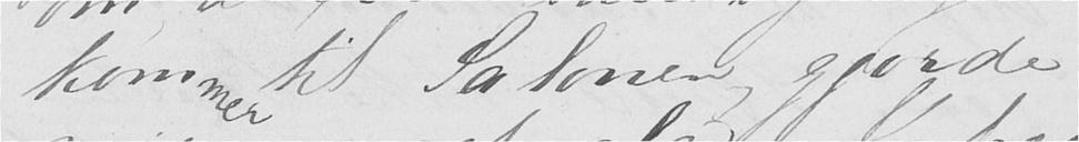kommer til Salonen, gjorde
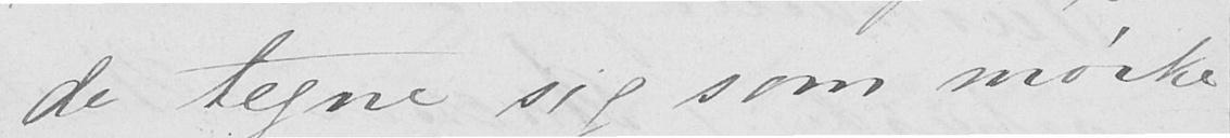de tegne sig som mørke
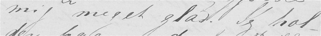mig meget glad. Jeg hol-
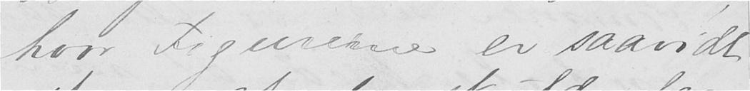hvor Figurerne er saavidt
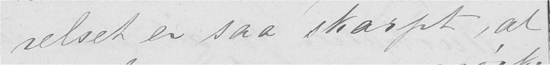relset er saa skarpt, at
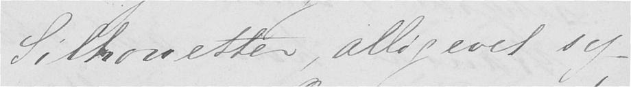Silhouetter, alligevel sy-
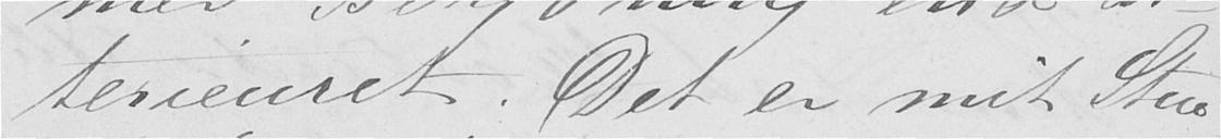terieuret. Det er mit Stue-
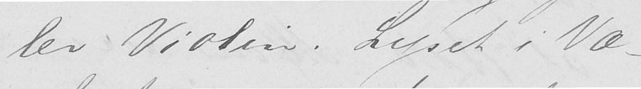ler Violin. Lyset i Væ-
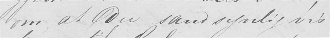om at Du sandsynligvis
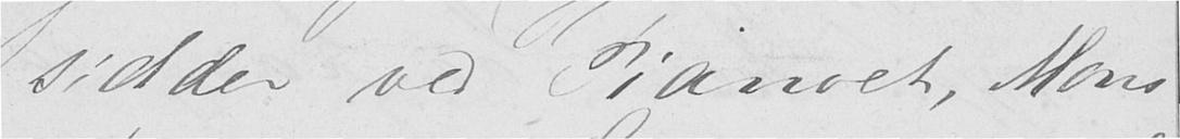sidder ved Pianoet, Mons
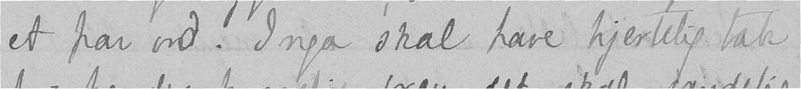et par ord. Inga skal have hjertelig tak
